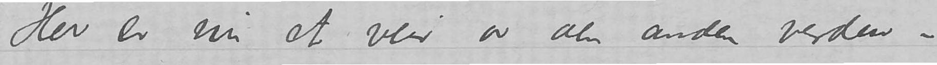Her er nu et veir av den anden verden –
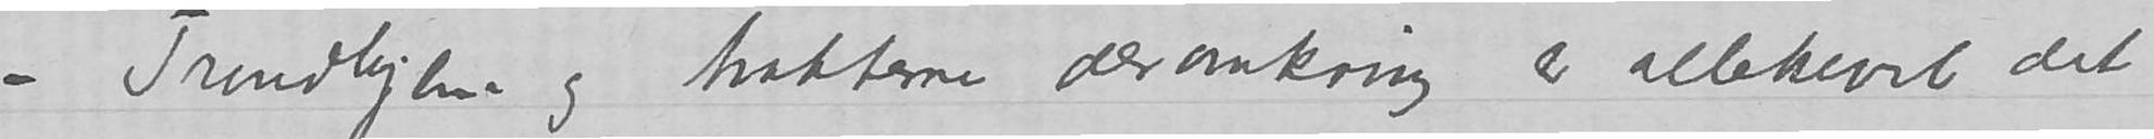Trondhjem og trakterne der omkring er allikevel det
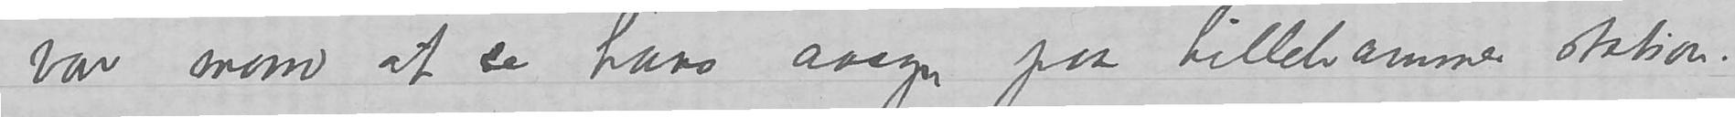var morro at se hans aasyn paa Lillehammer station.
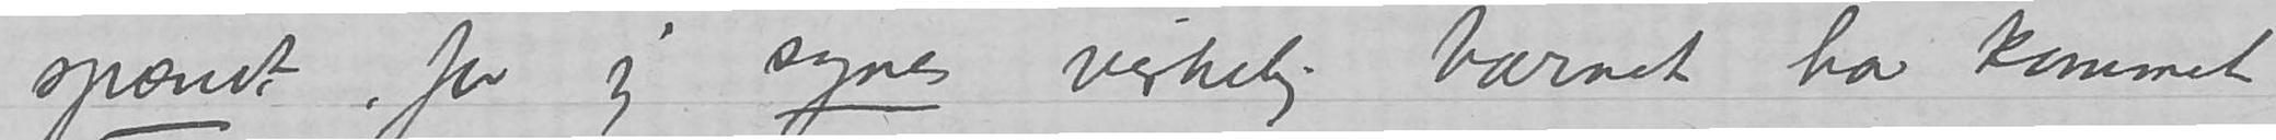spændt, for jeg synes virkelig barnet har kommet
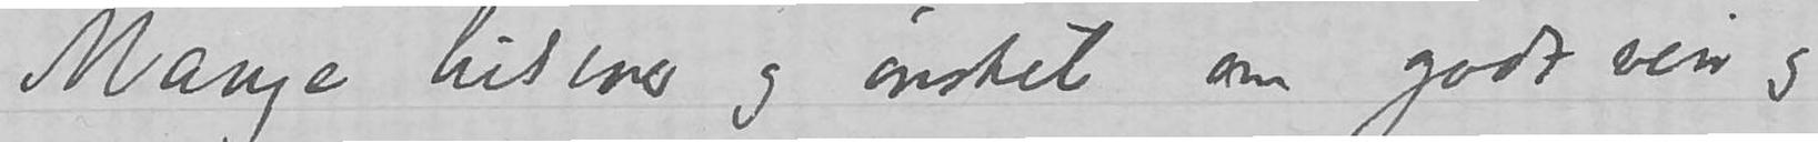Mange hilsener og ønsket om godt veir og
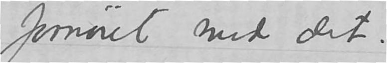fornøiet med det.
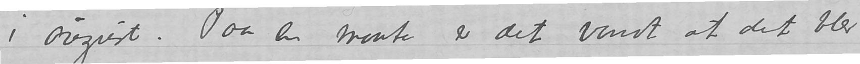i august.  Paa en maate er det vondt at det blev
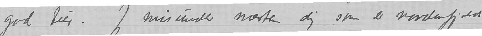god tur.. Jeg misunder næsten dig som er nordenfjelds
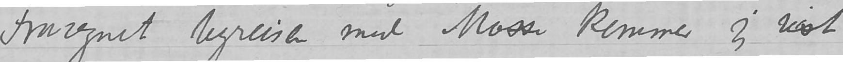Fraregnet togreisen med Mosse kommer jeg vist
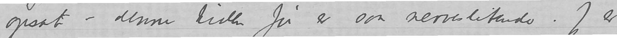opsat – denne tiden før er saa nerveslitende.  Jeg er
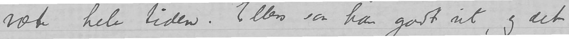væte hele tiden.  Ellers saa han godt ut, og det
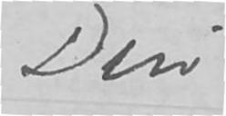Din
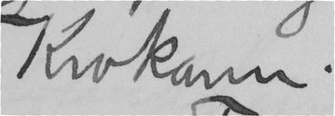Krokann.
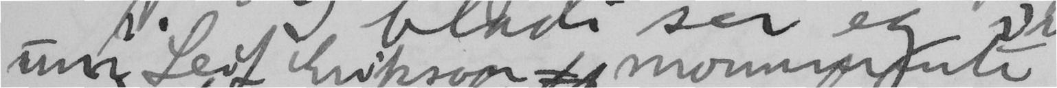Um Leif Eirikson-monumente
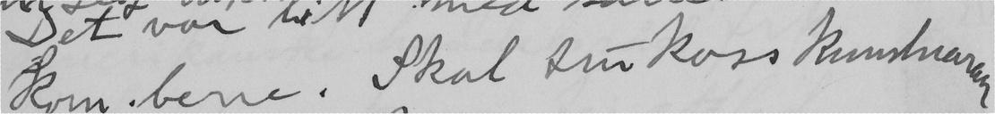kom berre. Skal tru koss kunstnaren
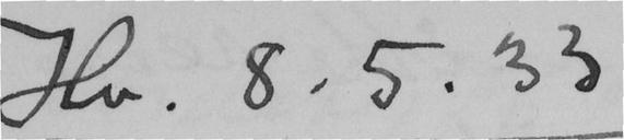Hv. 8.5.33
